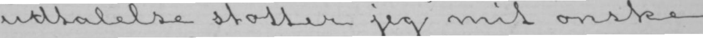udtalelse støtter jeg mit ønske
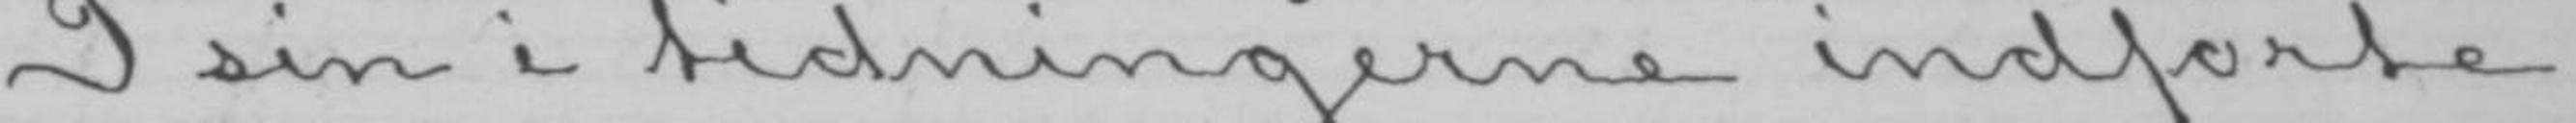I sin i tidningerne indførte
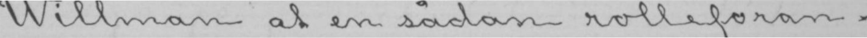Willman at en sådan rolleforan-
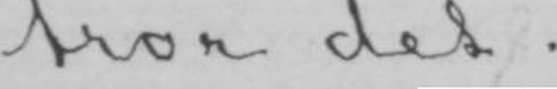tror det.
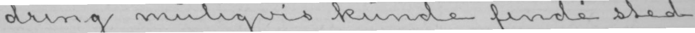dring muligvis kunde finde sted
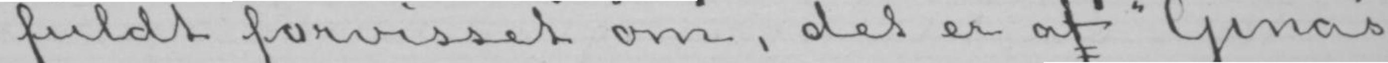fuldt forvisset om, det er aft «Gina»s
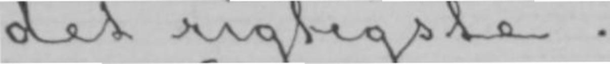det rigtigste.
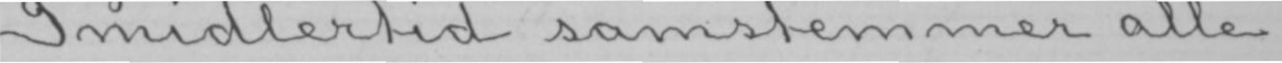Imidlertid samstemmer alle
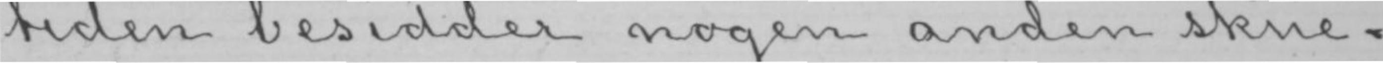tiden besidder nogen anden skue-
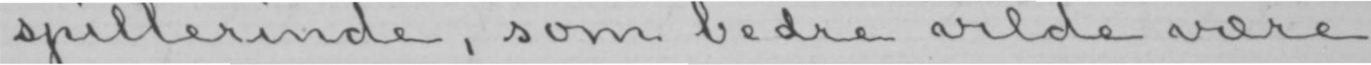spillerinde, som bedre vilde være
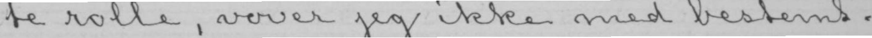te rolle, vover jeg ikke med bestemt-
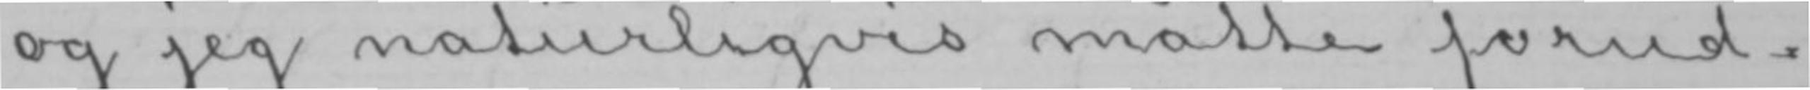og jeg naturligvis måtte forud-
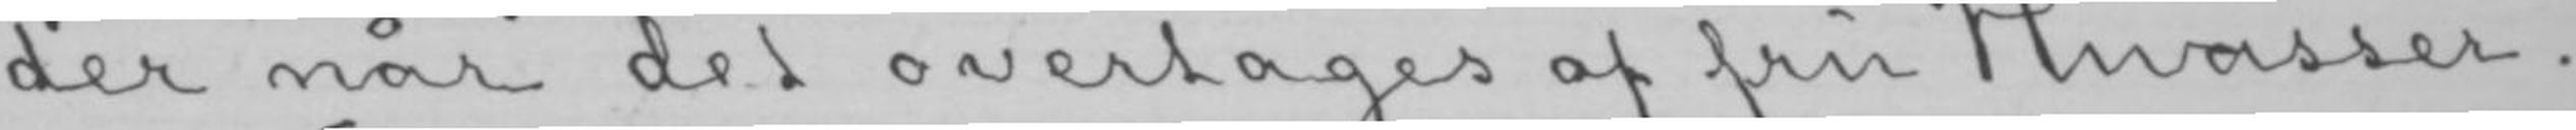der når det overtages af fru Hwasser.
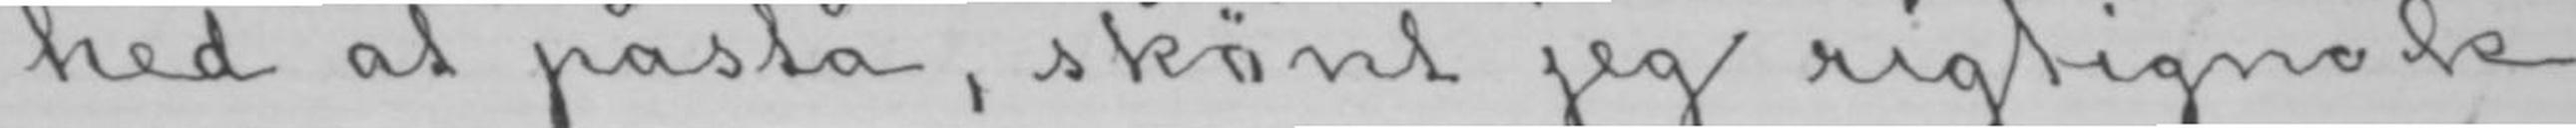hed at påstå, skønt jeg rigtignok
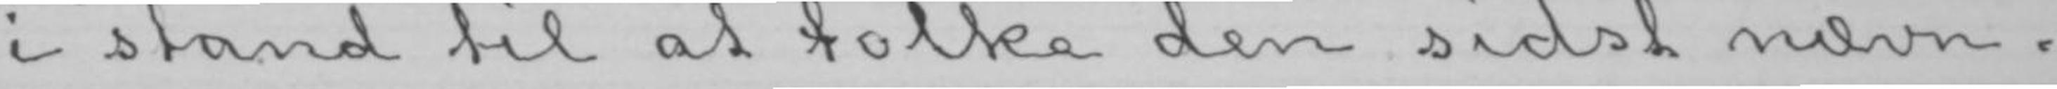i stand til at tolke den sidst nævn-
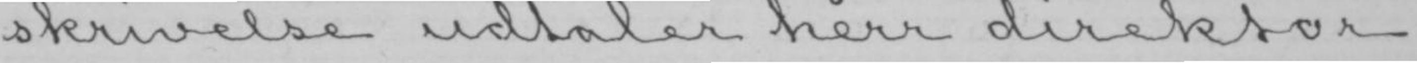skrivelse udtaler herr direktør
